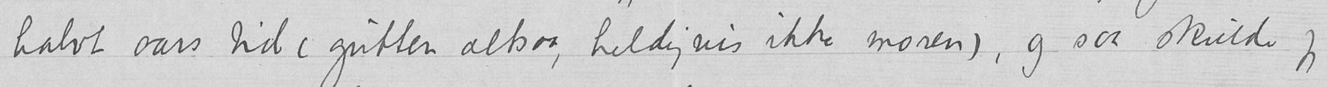halvt aars tid (gutten altsaa, heldigvis ikke moren), og saa skulde jeg
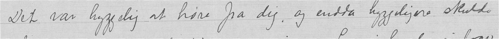Det var hyggelig at høre fra dig, og endda hyggeligere skulde
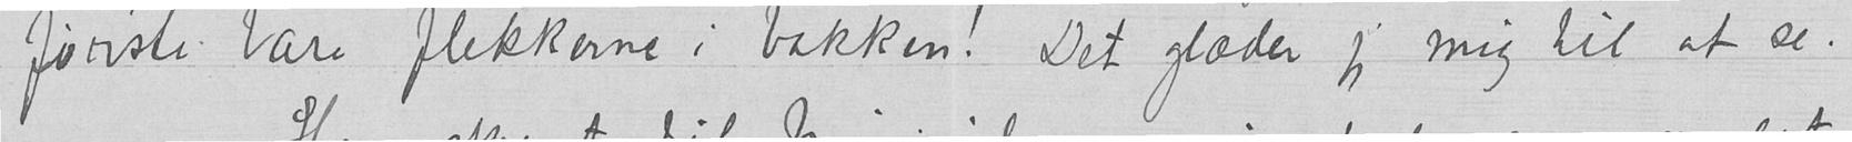første bare flekkerne i bakken! Det glæder jeg mig til at se.
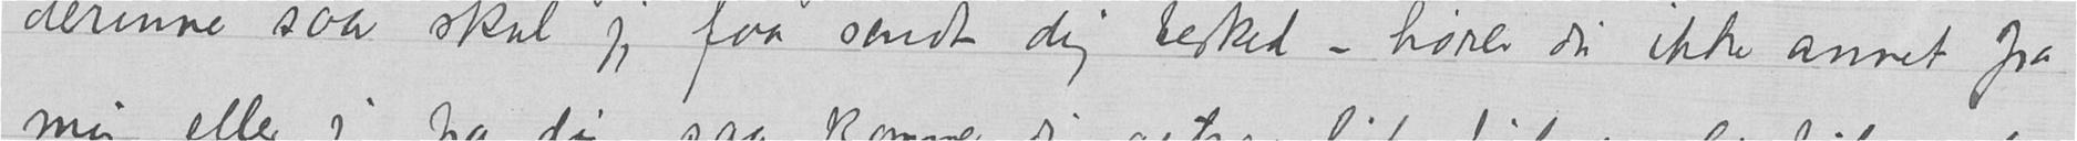derinne saa skal jeg faa sendt dig besked – hører du ikke annet fra
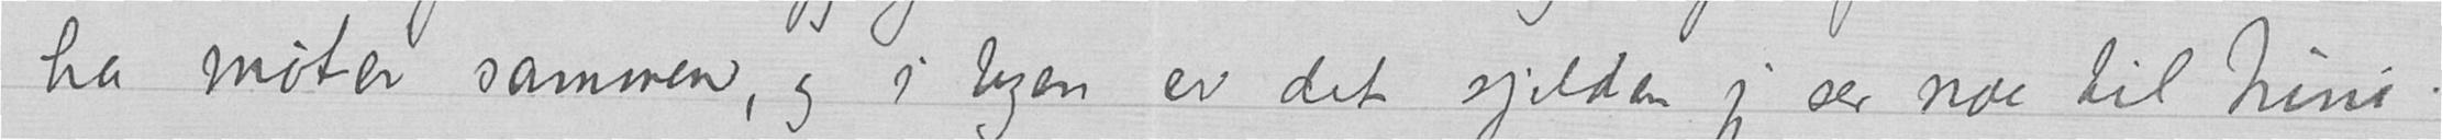ha møter sammen, og i byen er det sjelden jeg ser noe til Nini.
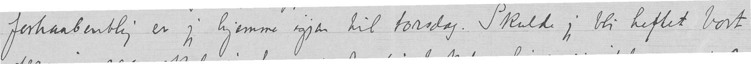forhaabentlig er jeg hjemme igjen til torsdag. Skulde jeg bli heftet bort
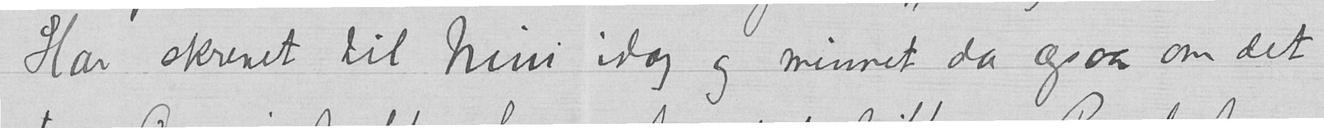Har skrevet til Nini idag og minnet da ogsaa om det
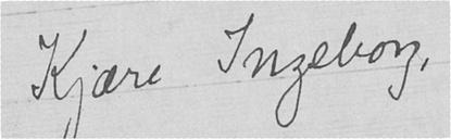Kjære Ingeborg,
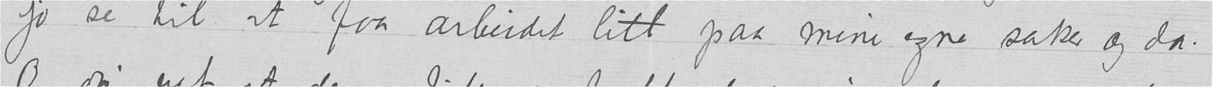jo se til at faa arbeidet litt paa mine egne saker og da.
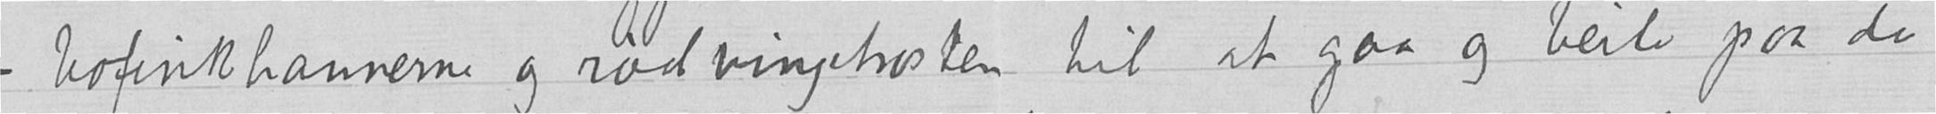- bofinkhannerne og rødvingetrosten til at gaa og beite paa de
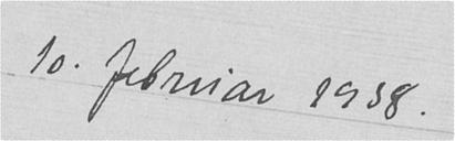10. februar 1938.
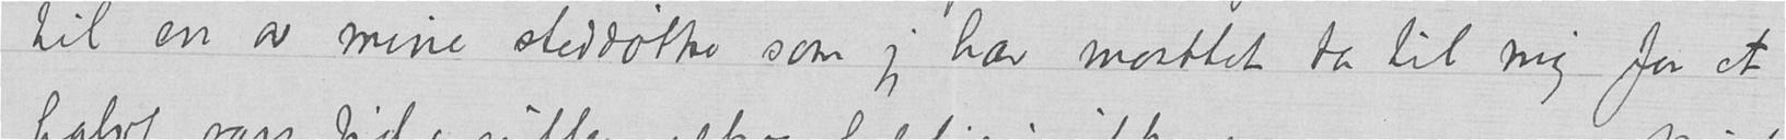til en av mine sleddøttre som jeg har maattet ta til mig for et
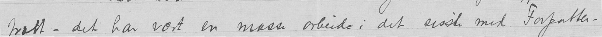trætt – det har vært en masse arbeide i det sisste med Forfatter-
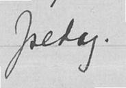fredag.
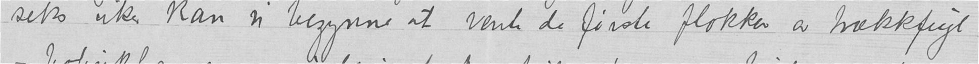seks uker kan vi begynne at vente de første flokker av trækkfugl
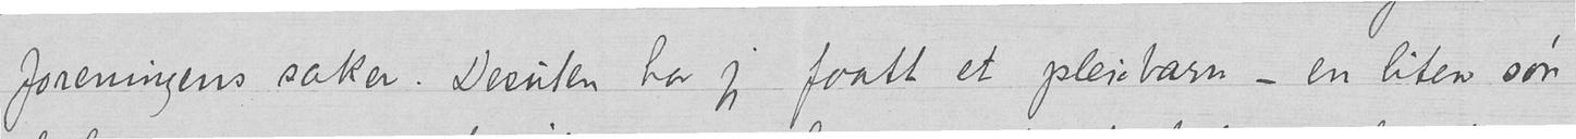foreningens saker. Desuten har jeg faatt et pleiebarn - en liten søn
